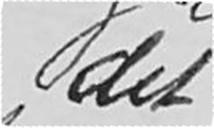det
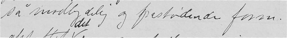så modbydelig og frastødende form.
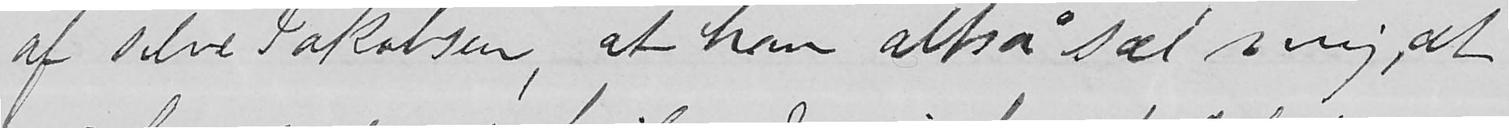af selve Jakobsen, at han altså sae' mig, at
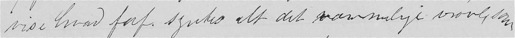vise hvad forf. syntes alt det væmmelige vrøvl, som
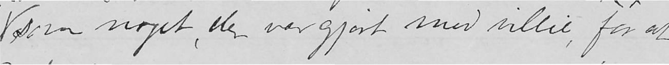som noget, der var gjort med villie, for at
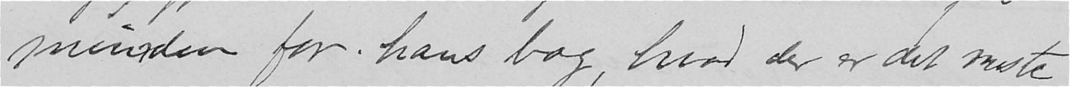munden for hans bog, hvad der er det værste
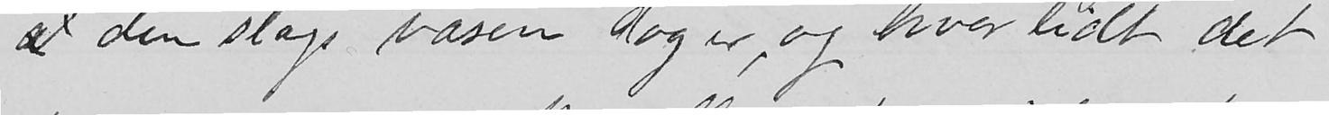al den slags væsen dog er, og hvor lidt det
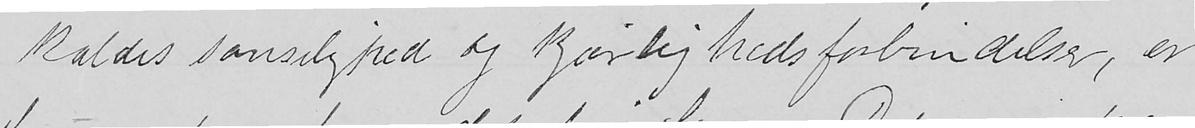kaldes sanselighed og kjærlighedsforbindelser, er
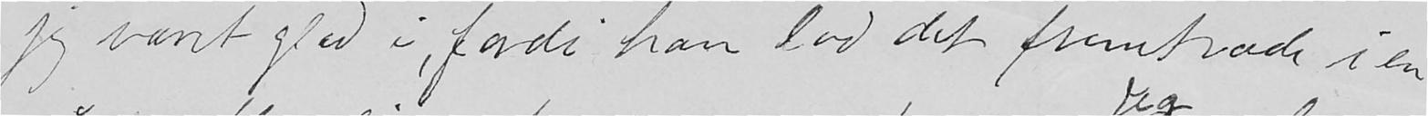jeg været glad i, fordi han lod det fremtræde i en
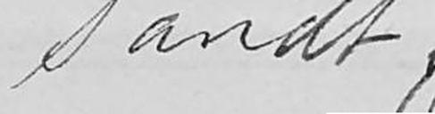sandt,
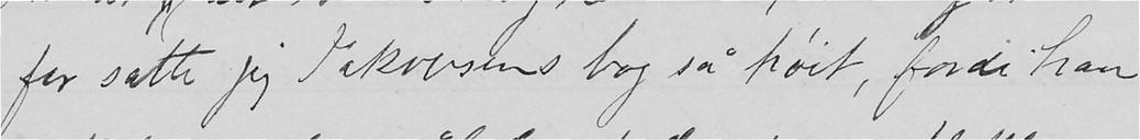for satte jeg Jakobsens bog så høit, fordi han
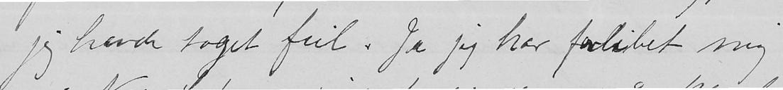jeg havde taget feil. Ja jeg har forlibet mig
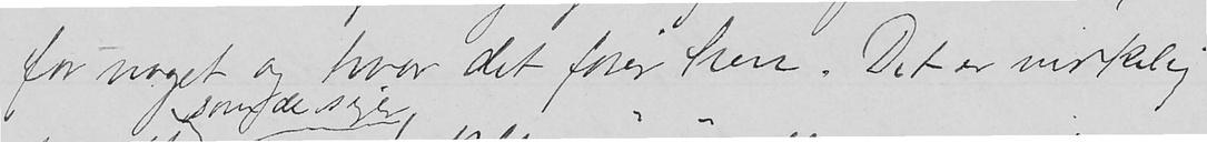for noget og hvor det fører hen. Det er virkelig
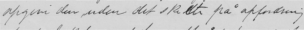opgive den, uden det skedte på opfordring
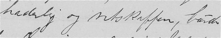hæderlig og retskaffen, burde
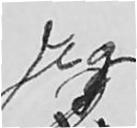Jeg
